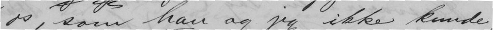os, som han og jeg ikke kunde,
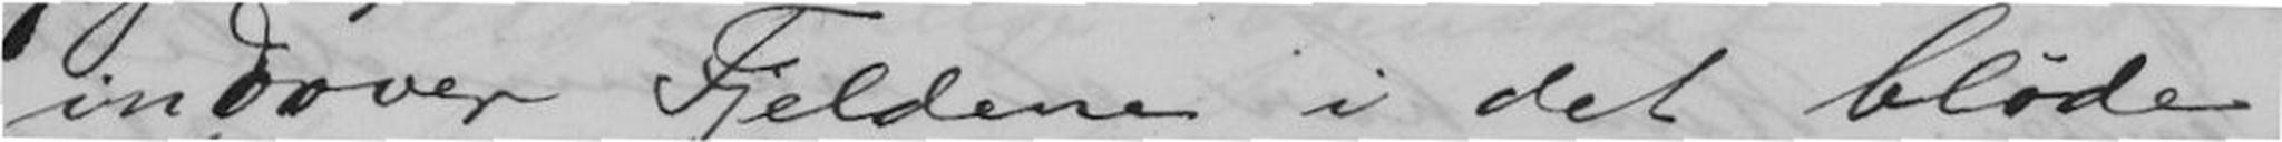indover Fjeldene i det bløde
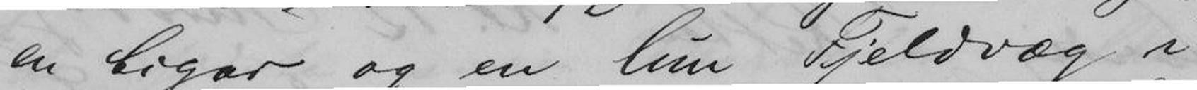en Cigar og en lun Fjeldvæg i
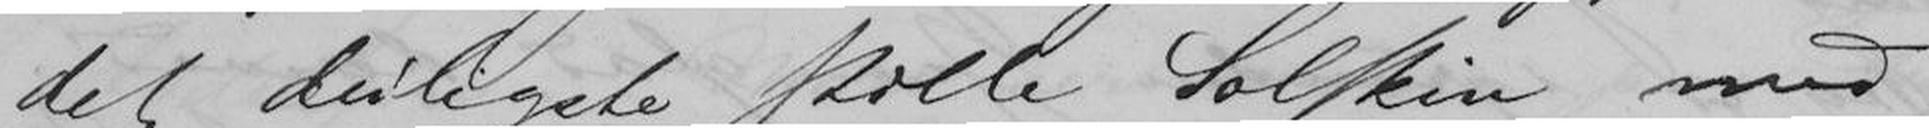det deiligste Stille Solskin med
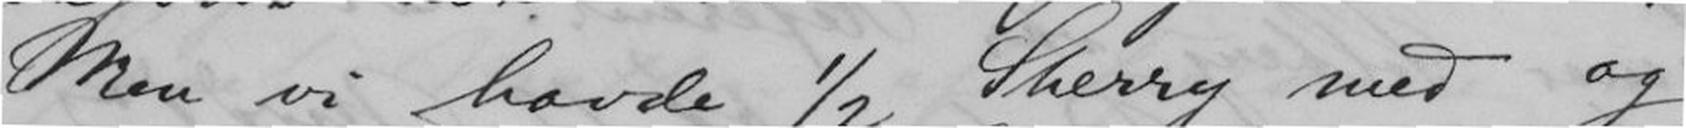Men vi havde ½ Sherry med og
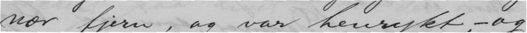nær fjern, og var henrykt, - og
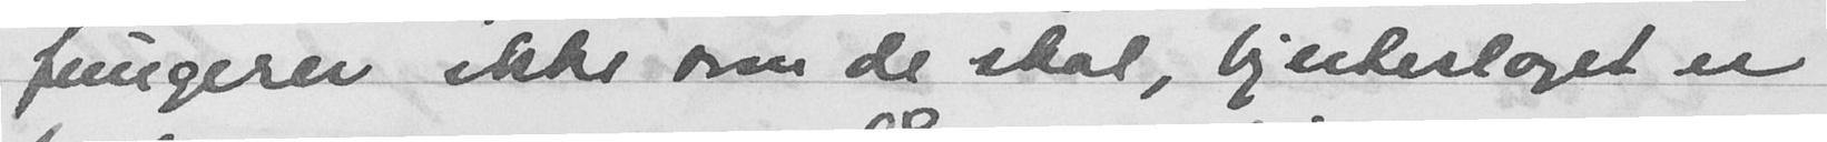fungerer ikke som de skal, hjerteslaget er
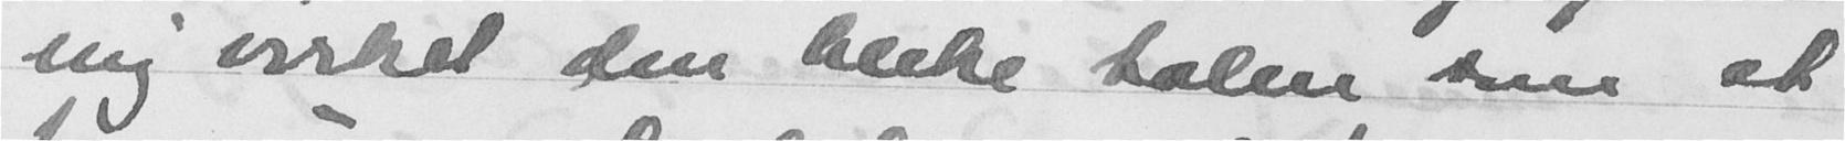mig virket den bleke talen som et
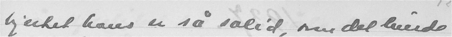hjertet hans er så solid, som det burde
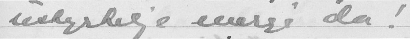ustyrtelige energi da!
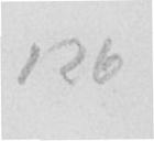126
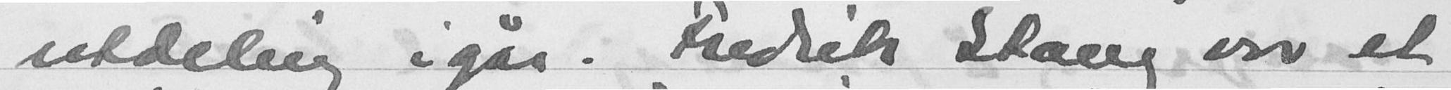utdeling igår. Fredrik Stang var et
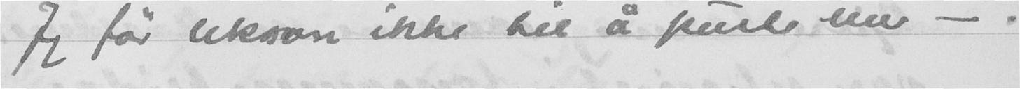Jeg får liksom ikke til å puste mer - .
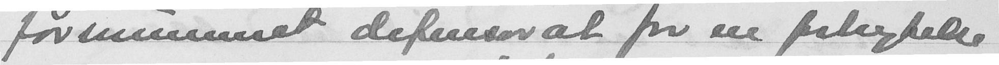forsmummet defensorat for en forlighelse
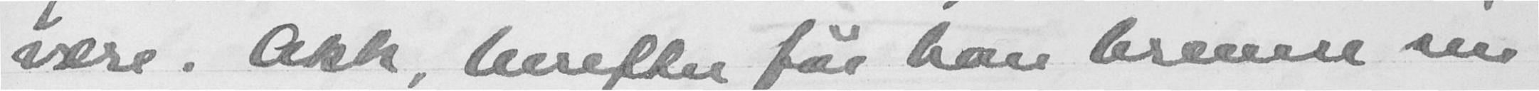være. Akk, herefter får han brenne sin
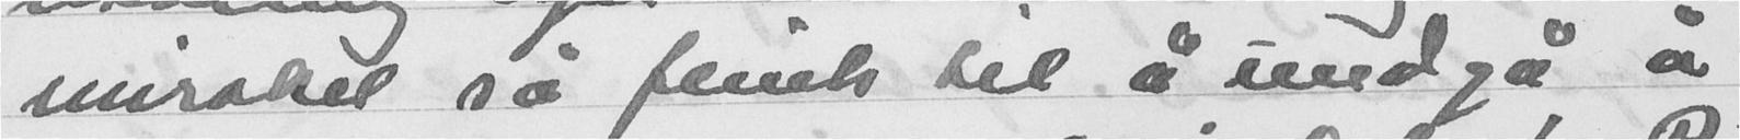mirakel så flink til å undgå å
